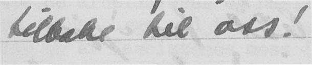tilbake til oss!
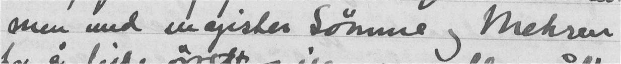men med magister Sømme og Mehren
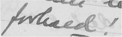forhold!
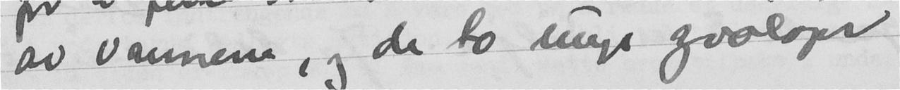av vannene, og de to unge geologer
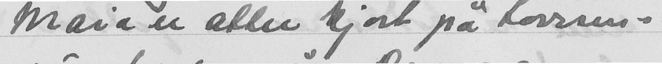Maia er atter kjørt på lovisen-
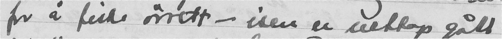for å fiske ørrett - isen er nettop gått
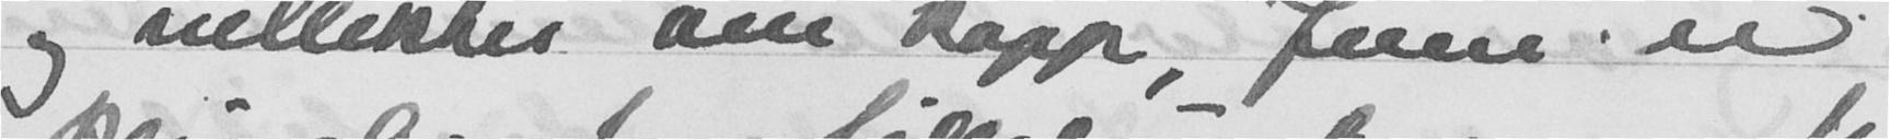og nellikker om kapp, Fuen er
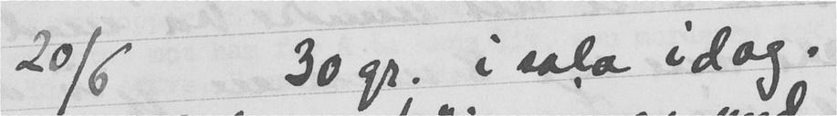20/6 30 gr. i sola idag.
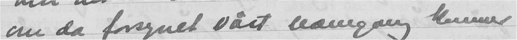om da forsynet vårt noengang kommer
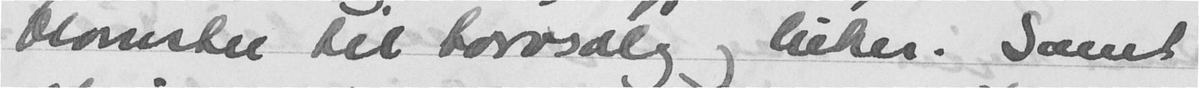blomster til torvsalg og leiker. Samt
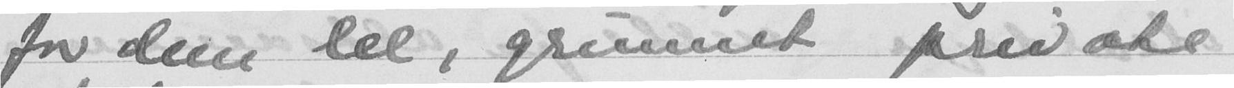for dem lel, grunnet private
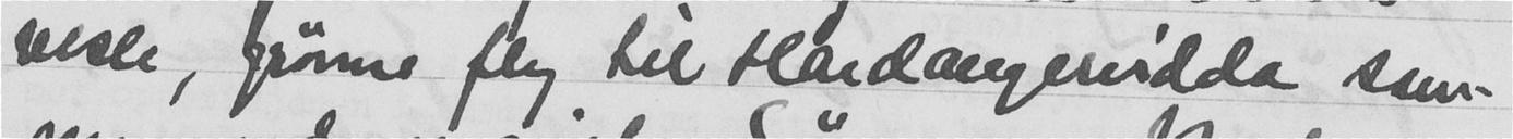vesle, grønne fly til Hardangervidda sam-
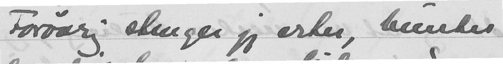Forvøvrig slenger jeg erter, bunter
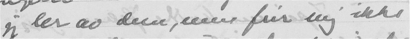jeg ler av dem, men frir mig ikke
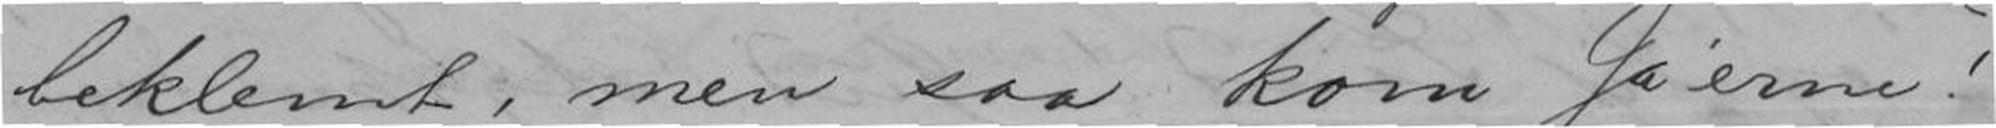beklemt, men saa kom Ja'erne!
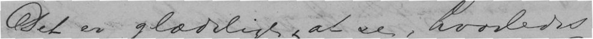Det er glædeligt, at se, hvorledes
